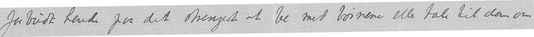forbudt hende paa det strengeste at be med børnene eller tale til dem om
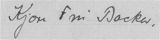Kjære Fru Backer,
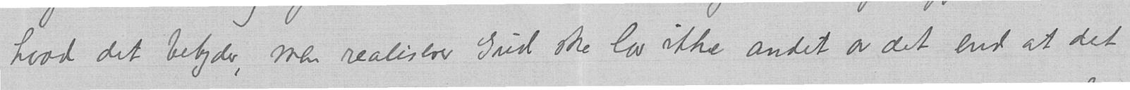hvad det betyder, men realiserer Gud ske lov ikke andet av det end at det
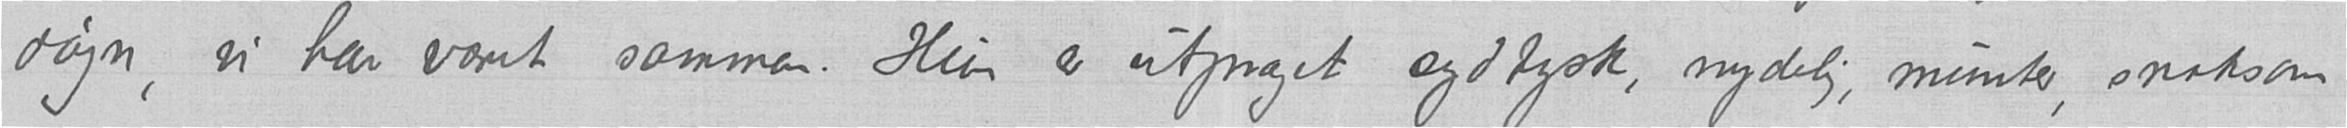døgn, vi har været sammen. Hun er utpræget sydtysk, nydelig, munter, snaksom
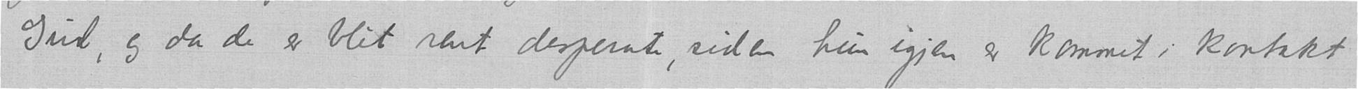Gud, og da de er blit rent desperate, siden hun igjen er kommet i kontakt
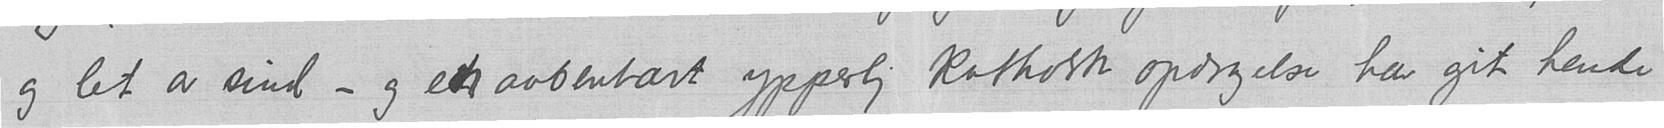og let av sind – og en saabenbart ypperlig katholsk opdragelse har git hende
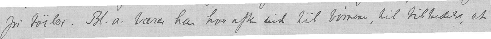fri tøiler. Bl.a. bærer han hver aften ind til børnene, til tilbedelse, et
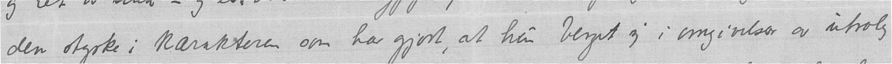den styrke i karakteren som har gjort, at hun berget sig i omgivelser av utrolig
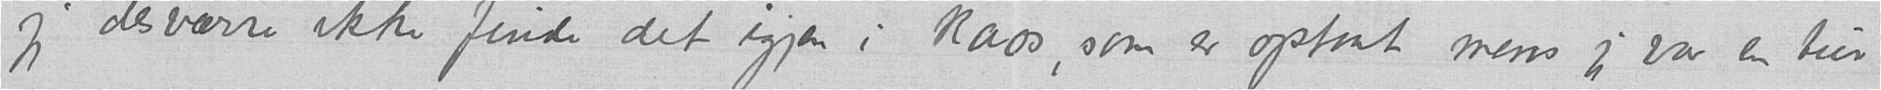jeg desværre ikke finde det igjen i kaos, som er opstaat mens jeg var en tur
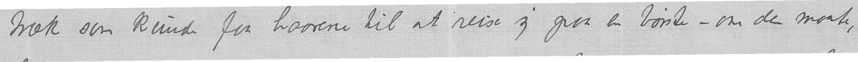træk som kunde faa haarene til at reise sig paa en børste – om den maate,
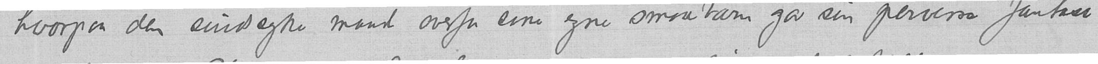hvorpaa den sindssyke mand overfor sine egne smaabarn gav sin perverse fantasi
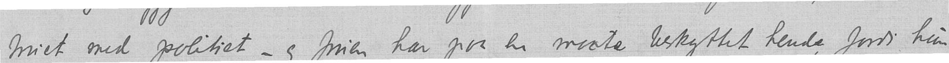truet med politiet – og fruen har paa en maate beskyttet hende fordi hun
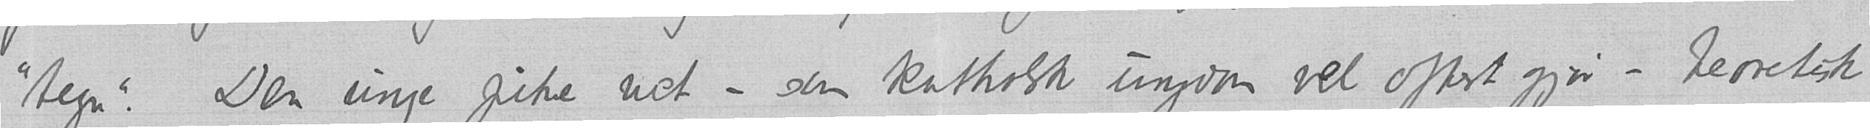"tegn". Den unge pike vet – som katholsk ungdom vel oftest gjør - teoretisk
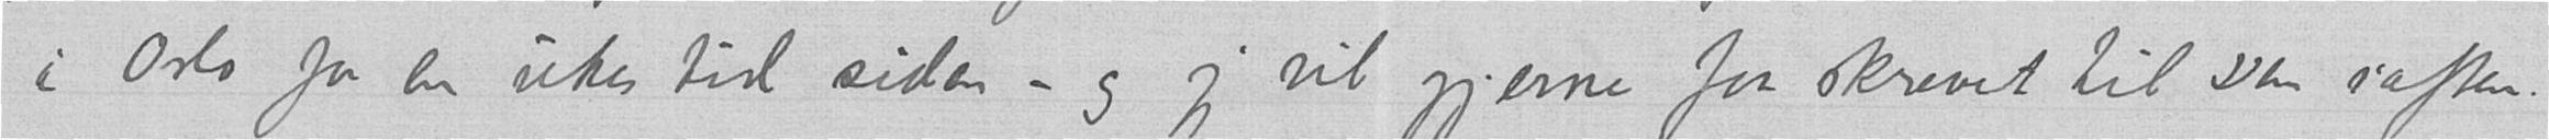i Oslo for en ukes tid siden – og jeg vil gjerne faa skrevet til Dem iaften.
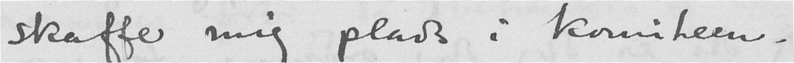skaffe mig plads i komiteen.
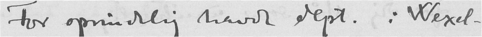For oprindlig havde dept. i Wexel-
